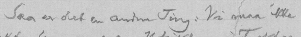Saa er det en anden Ting: Vi maa ikke
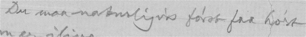Du maa naturligvis først faa hørt
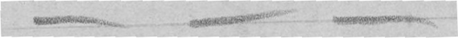- - -
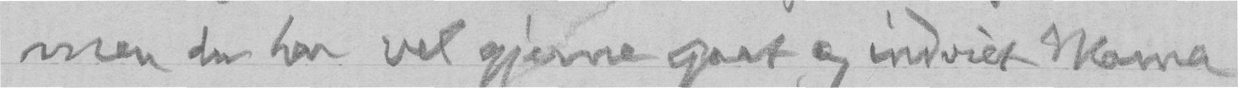men du har vel gjerne gaat og indviet Mama
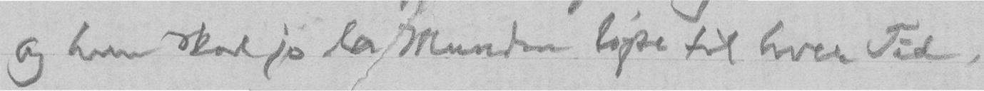og hun skal jo la Munden løpe til hver Tid.
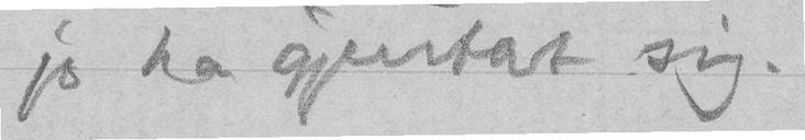jo ha gjentat sig.
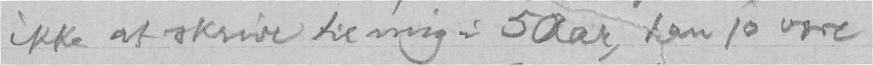ikke at skrive til mig i 5 Aar, kan jo være
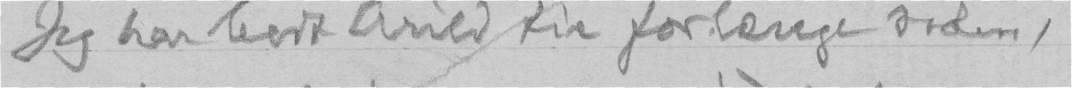Jeg har bedt Arild tie forlænge siden,
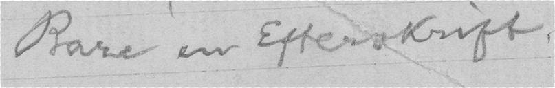Bare en Efterskrift,
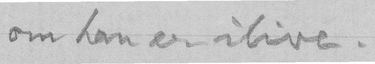om han er ilive.
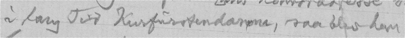i lang Tid Kurfürstendamm, saa blev han
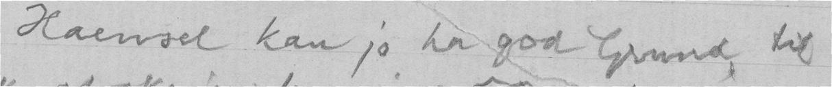Haensel kan jo ha god Grund til
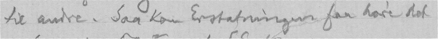til andre. Saa kan Erstatningen faa høre det
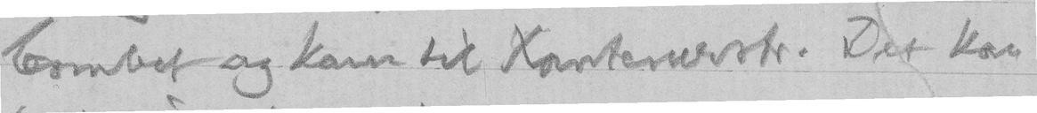bombet og kom til Xantenerstr. Det kan
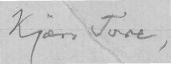Kjære Tore,
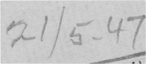21/5. 47
